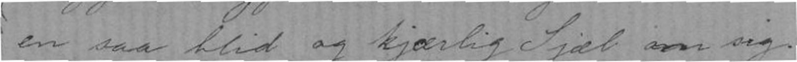en saa blid og kjærlig Sjæl om sig.
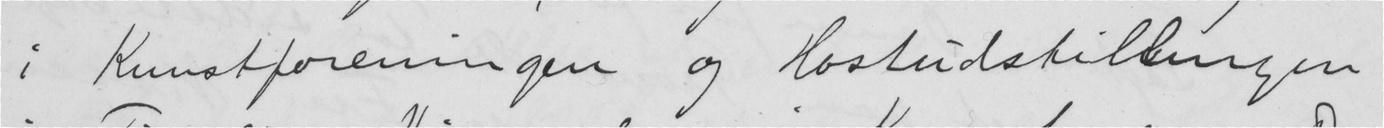i Kunstforeningen og Høstudstillingen
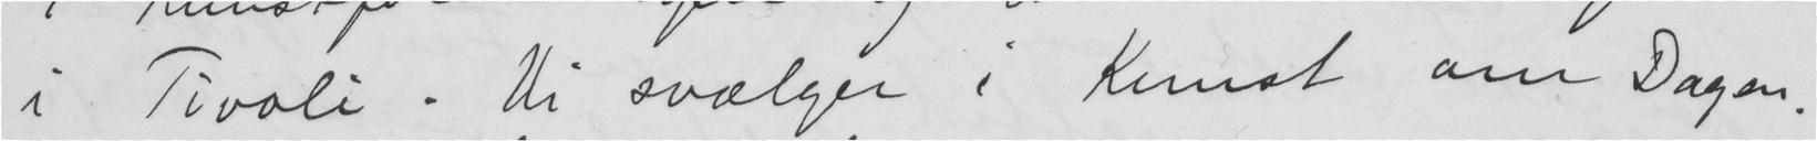i Tivoli. Vi svælger i Kunst om Dagen.
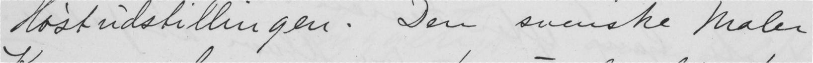Høstudstillingen. Den svenske Maler
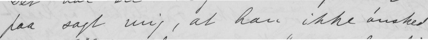faa sagt mig, at han ikke ønsked
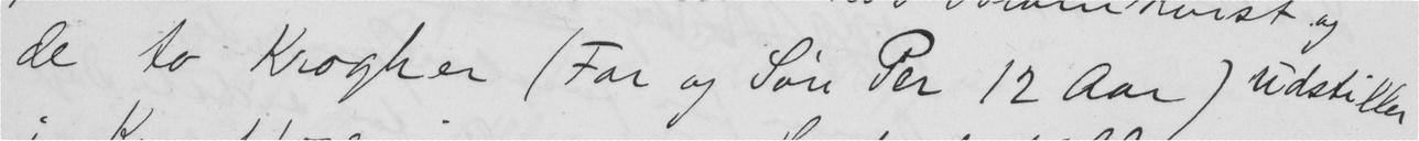de to Krogher (Far og Søn Per 12 Aar) udstiller
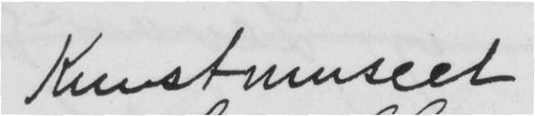Kunstmuseet
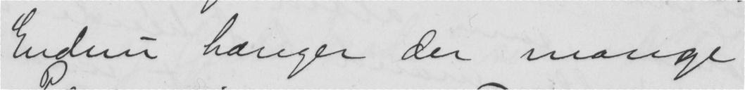Endnu hænger der mange
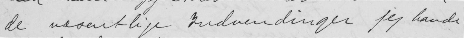de væsentlige Indvendinger jeg havde
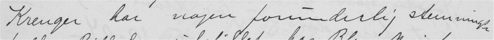Krenger har nogen forunderlig stemnings-
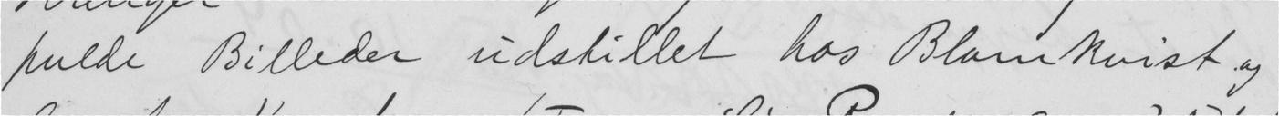fulde Billeder udstillet hos Blomkvist og
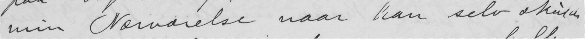min Nærværelse naar han selv skulde
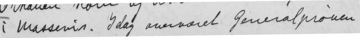i massevis. Idag overværet Generalprøven
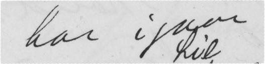har igaar
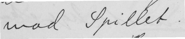mod Spillet.
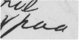paa
